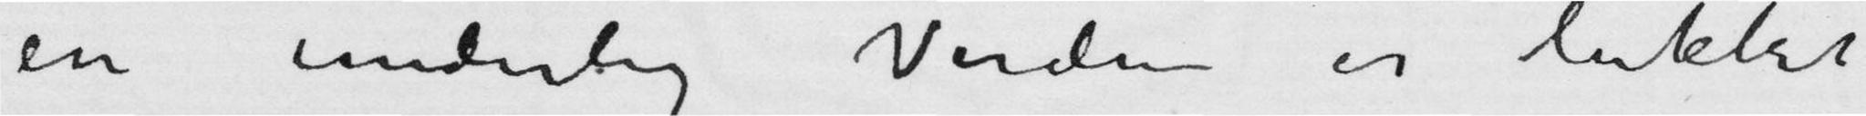en underlig Verden er lukket
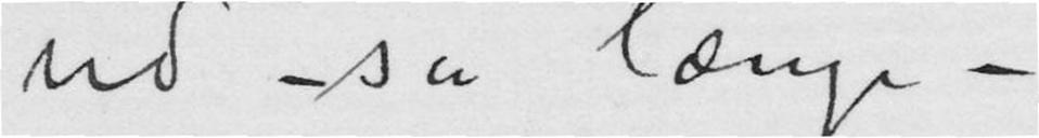ud – så længe –
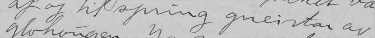af og til spring gneistar av
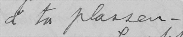å ta plassen –
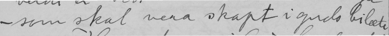– som skal vera skapt i guds bilæte
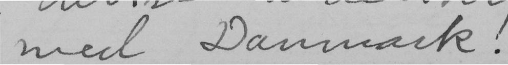med Danmark!
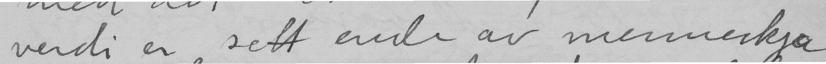verdi er sett ende av menneskja
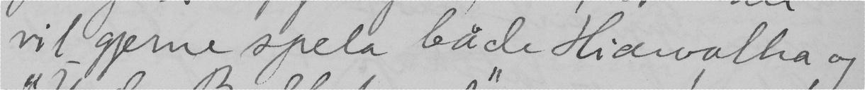vil gjerne spela både Hiawatha og
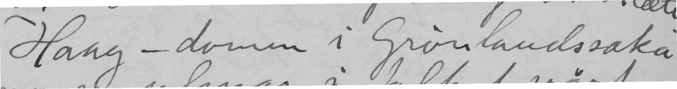Haag-domen i Grönlandssaka
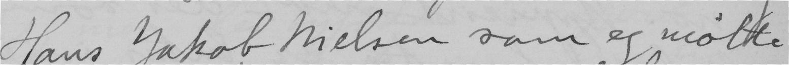Hans Jakob Nielsen som eg mötte
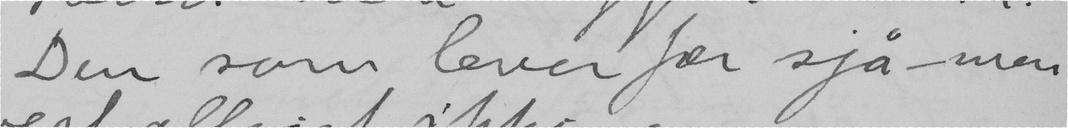Den som lever fær sjå – men
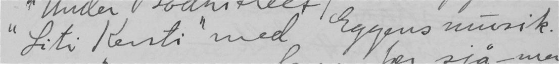"Liti Kersti" med Eggens musik.
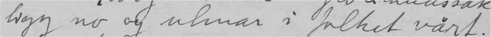ligg no og ulmar i folket vårt.
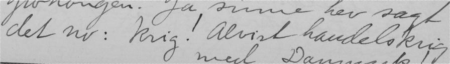det no: krig! Alvist handelskrig
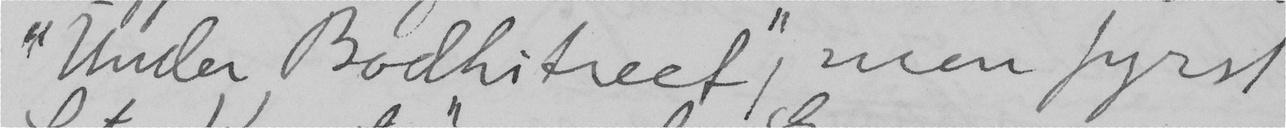"Under Bodhitreet", men fyrs
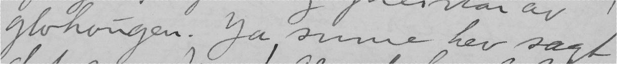glohougen. Ja summe hev sagt
